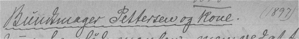Bundsmager Pettersen og kone. (1897)
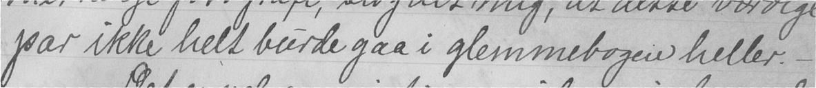par ikke helt burde gaa i glemmebogen heller. -
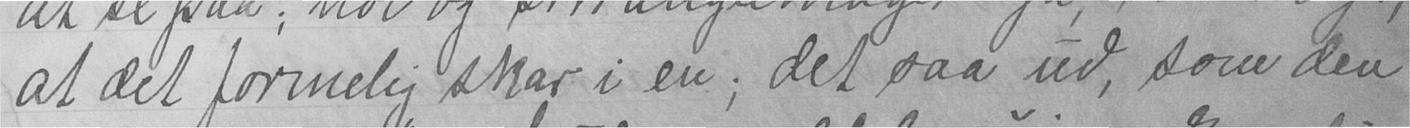at det formelig skar i en; det saa ud, som den
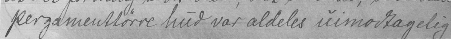pergamenttørre hud var aldeles uimodtagelig
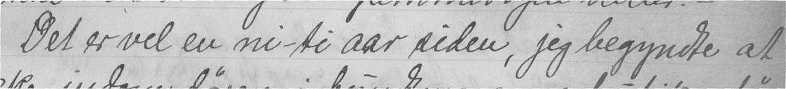Det er vel en ni- ti aar siden, jeg begyndte at
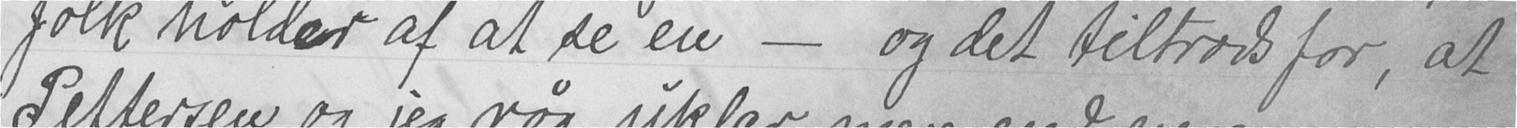folk holder af at se en - og det tiltrods for, at
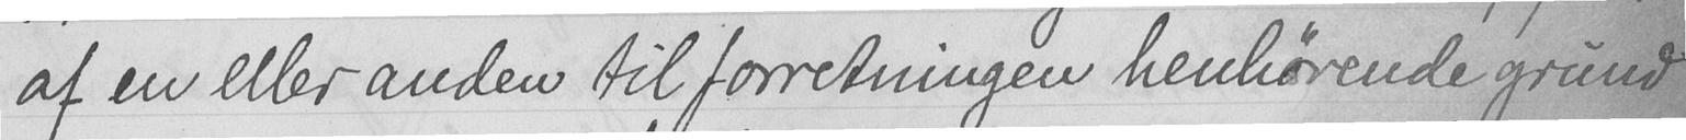af en eller anden til forretningen henhørende grund
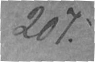207.
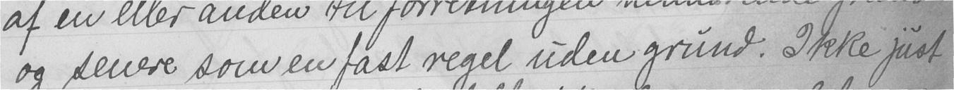og senere som en fast regel uden grund. Ikke just
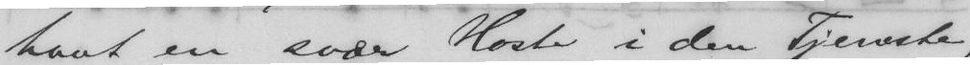havt en svær Hoste i den Tjeneste,
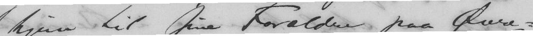hjem til sine Forældre paa Øvre-
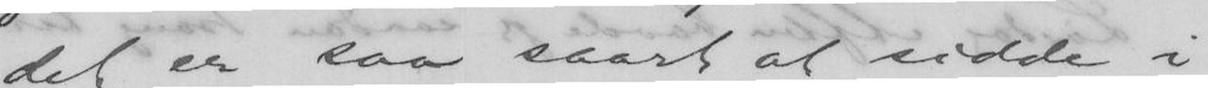det er saa saart at sidde i
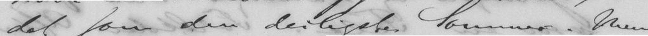det som den deiligste Sommer. Men
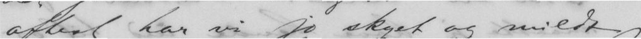oftest har vi jo skyet og mildt
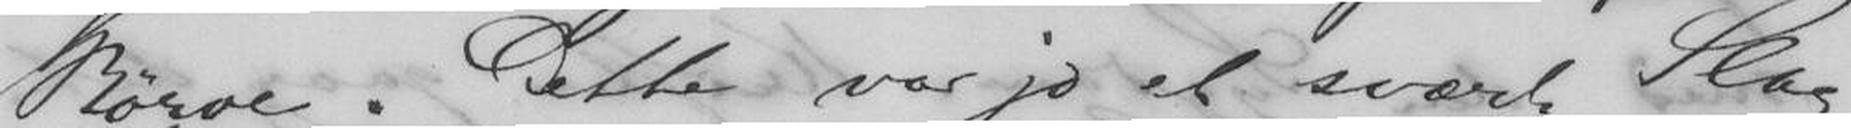Børve. Dette var jo et svært Slag
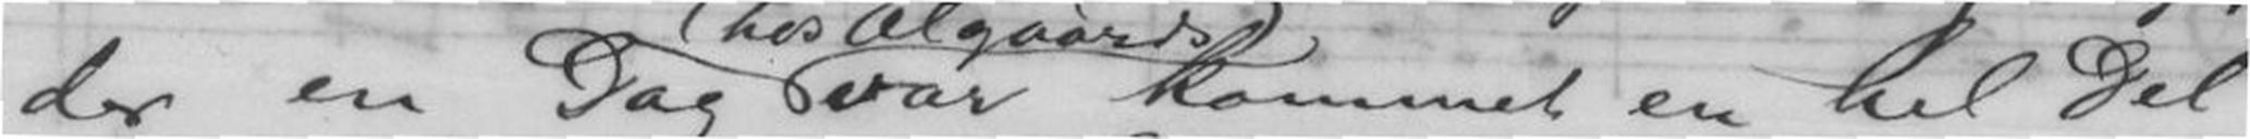der en Dag var kommet en hel Del
